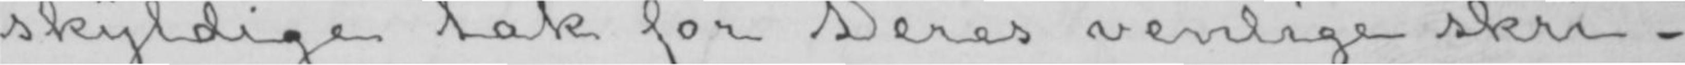skyldige tak for Deres venlige skri-
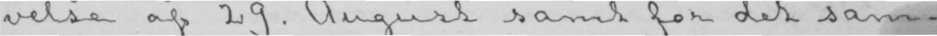velse af 29. August samt for det sam-
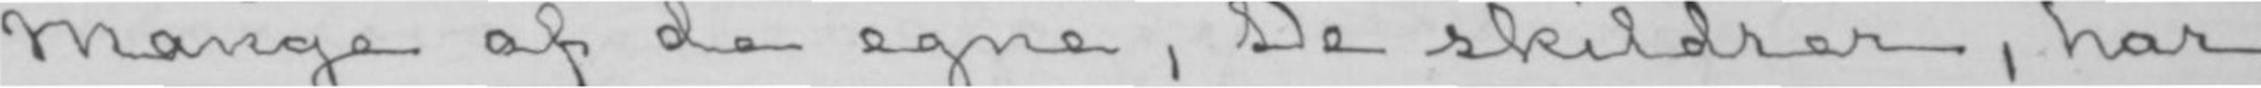Mange af de egne, De skildrer, har
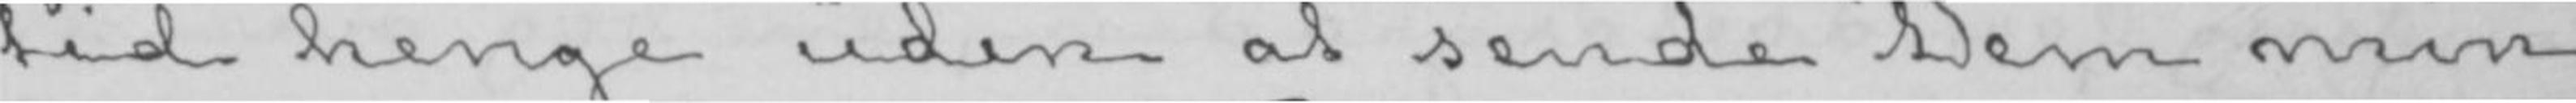tid henge uden at sende Dem min
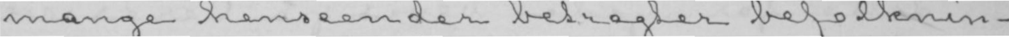mange henseender betragter befolknin-
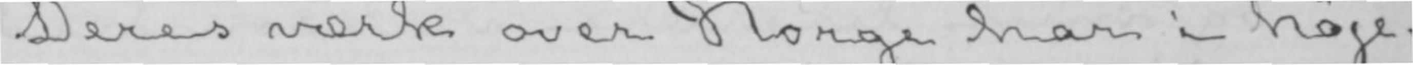Deres værk over Norge har i høje-
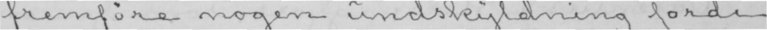fremføre nogen undskyldning fordi
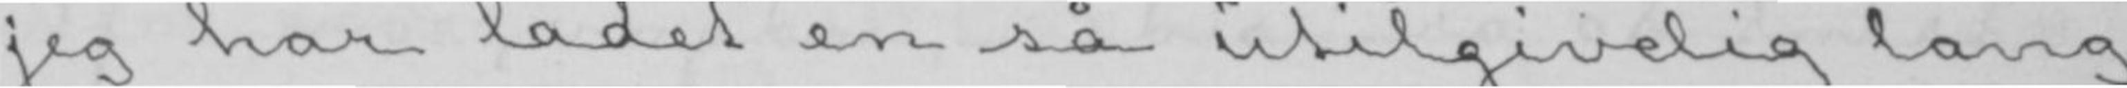jeg har ladet en så utilgivelig lang
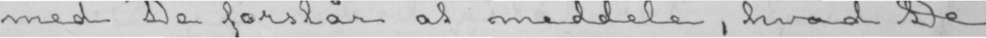med De forstår at meddele, hvad De
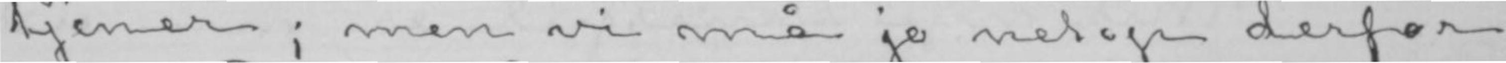tjener; men vi må jo netop derfor
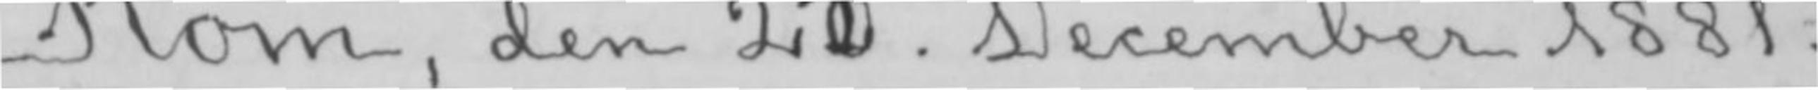Rom, den 20. December 1881.
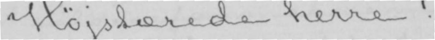Højstærede herre!
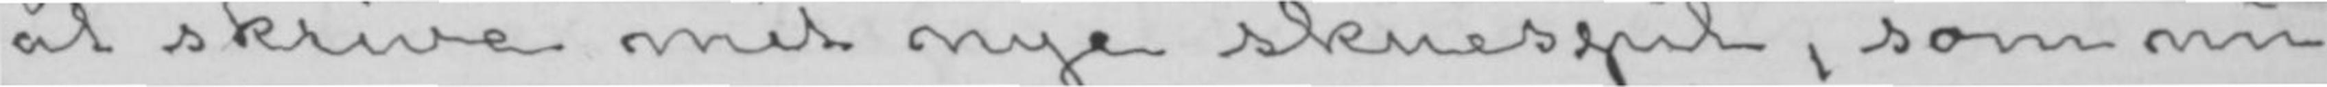at skrive mit nye skuespil, som nu
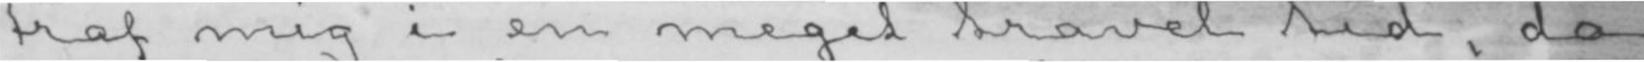traf mig i en meget travel tid, da
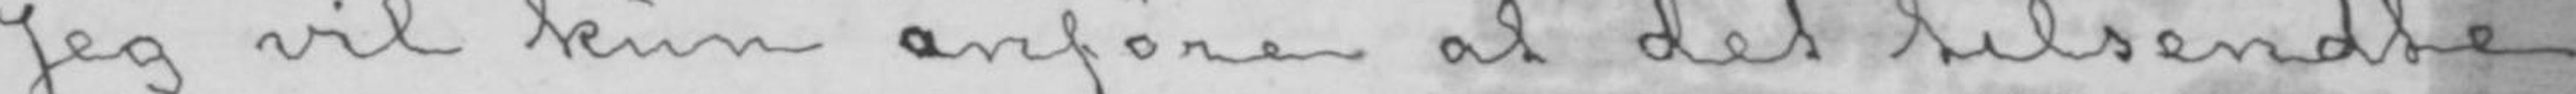Jeg vil kun anføre at det tilsendte
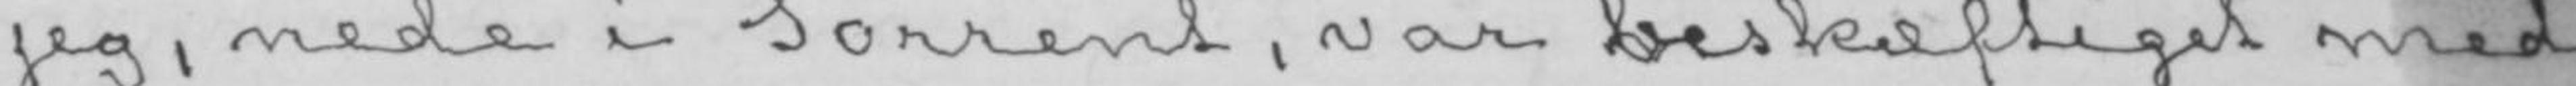jeg, nede i Sorrent, var beskæftiget med
